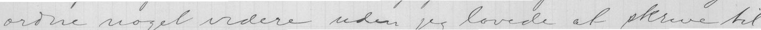ordne noget videre uden jeg lovede at skrive til
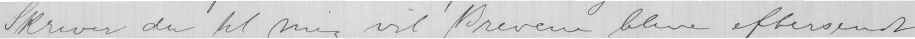Skriver du til mig vil Brevene blive eftersendt
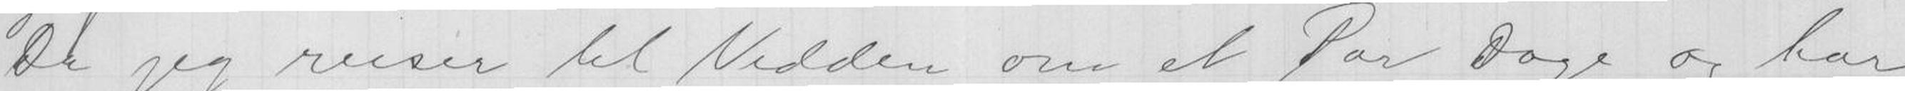Da jeg reiser til Vidden om et Par Dage og har
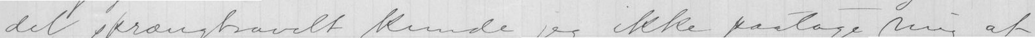det sprængtravelt kunde jeg ikke paatage mig at
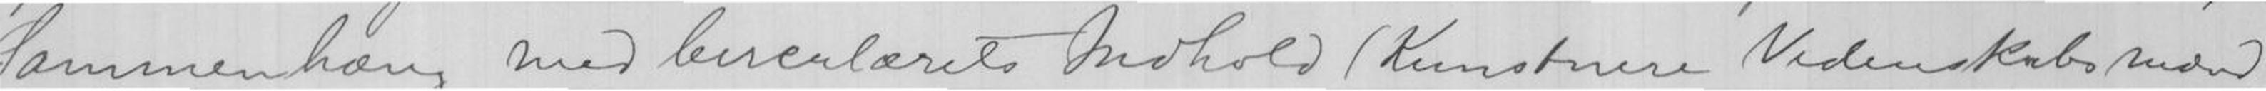Sammenhæng med Circulærets Indhold (Kunstnere Videnskabs mænd
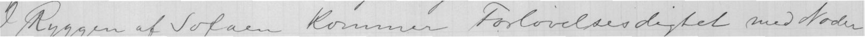I Ryggen af Sofaen kommer Forlovelsesdigtet med Noder
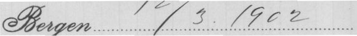Bergen 12/3. 1902
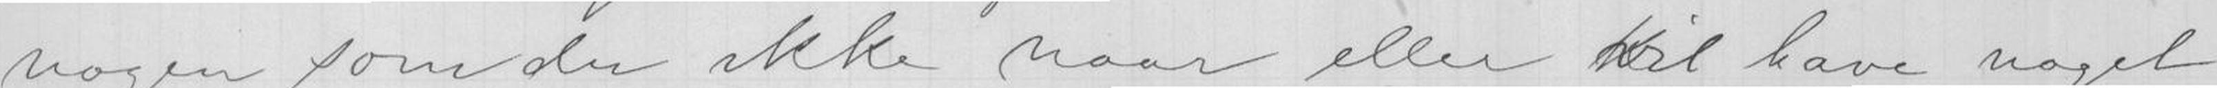nogen som du ikke naar eller vil have noget
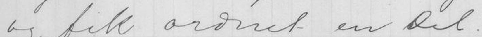og fik ordnet en Del.
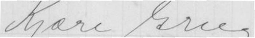Kjære Grieg
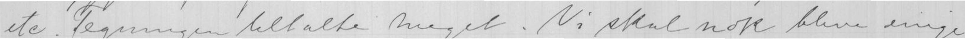etc. Tegningen tiltalte meget. Vi skal nok blive enige
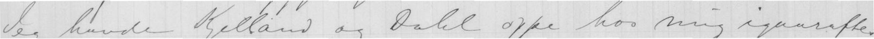Jeg havde Kjelland og Dahl oppe hos mig igaaraftes
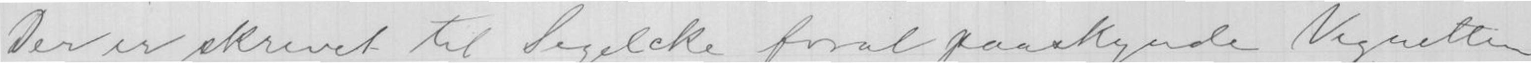Der er skrevet til Segelcke forat paaskynde Vegretten
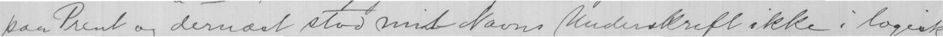paa Prent og dernæst stod mit Navns Underskrift ikke i logisk
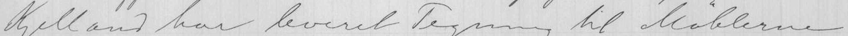Kjelland har leveret Tegning til Møblerne
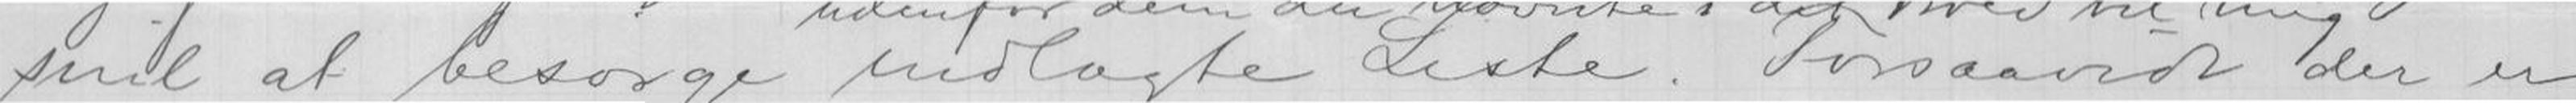snil at besørge indlagte Liste. Forsaavidt der er
